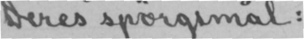Deres spørgsmål:
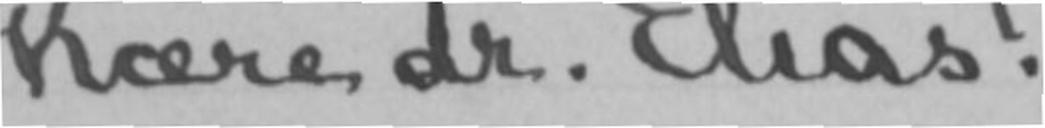Kære dr. Elias!
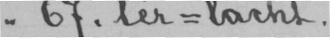" 67. lér = lacht.
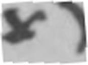*)
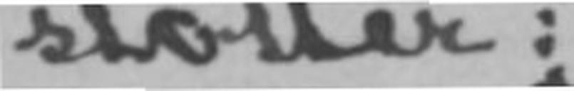støtter:
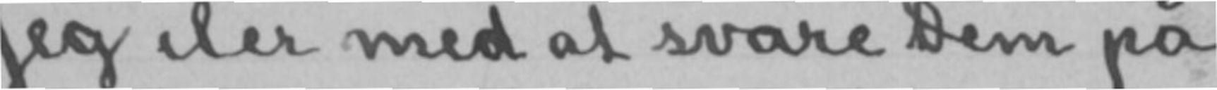Jeg iler med at svare Dem på
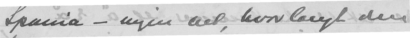Spania - ingen vet, hvor langt den
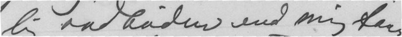lig indbuder end mig faar
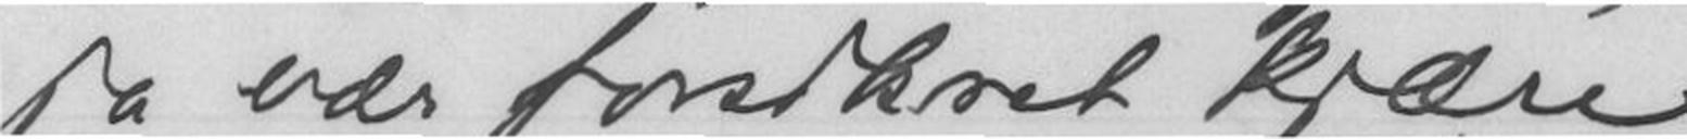ja vær forsikret kjære
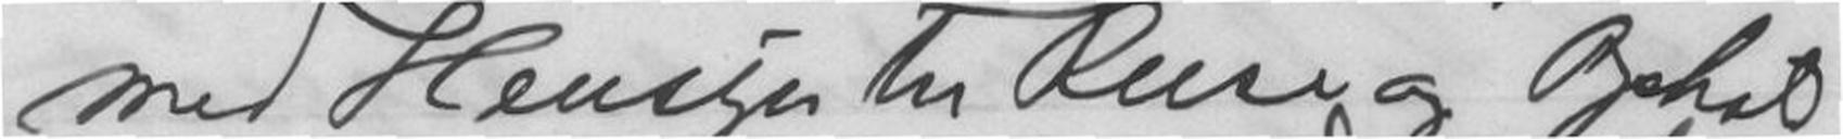med Hensyn til Reise og Ophold
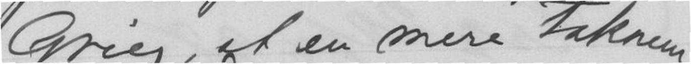Grieg, at en mere Taknem-
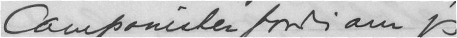Componister fordi om jeg
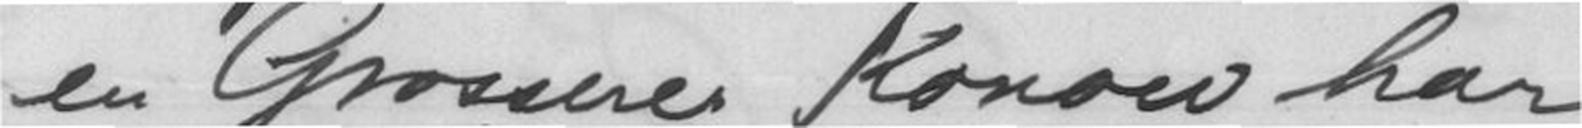en Grosserer Konow har
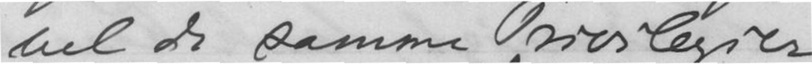vel de samme Privilegier
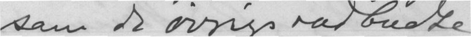som de øvrige indbudte
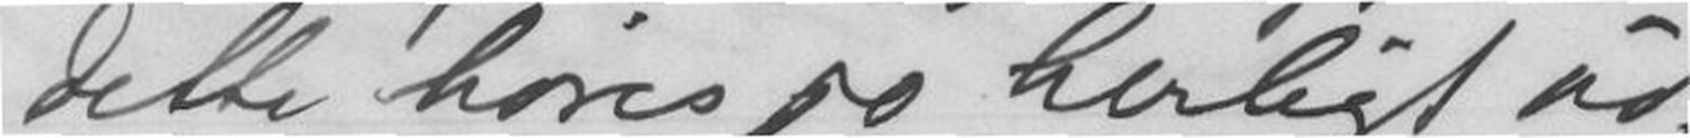dette høres jo herligt ud,
